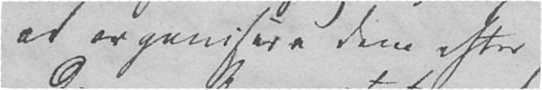at organisere dem efter
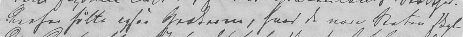Derfor følte ogsaa Grækerne, hvad de vare Staten skyl-
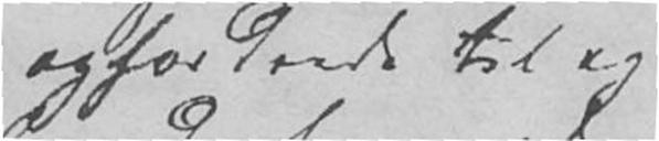opfordrede til og
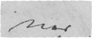nes
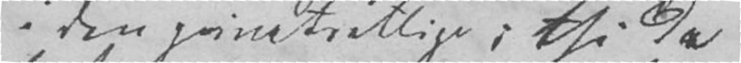i den privatretlige; thi da
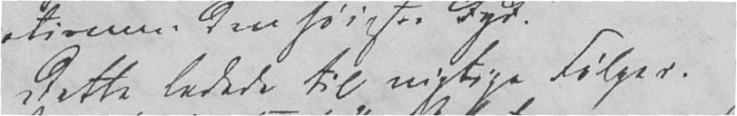Dette ledede til vigtige Følger.
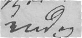endog
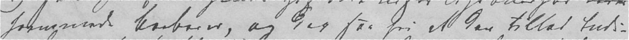fremmede Barbarer, og dog saa fri at den tillod Indi-
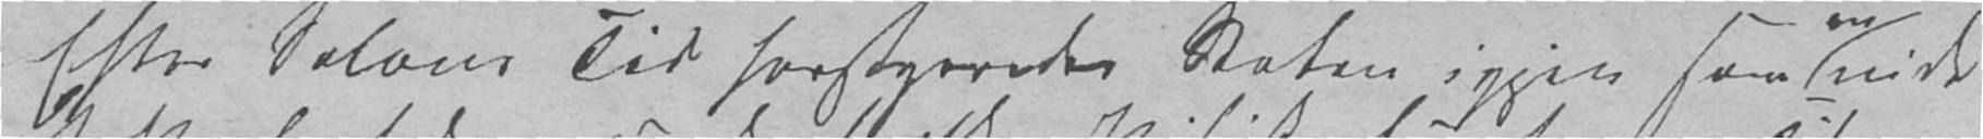Efter Solons Tid forstyrredes Staten igjen som unde
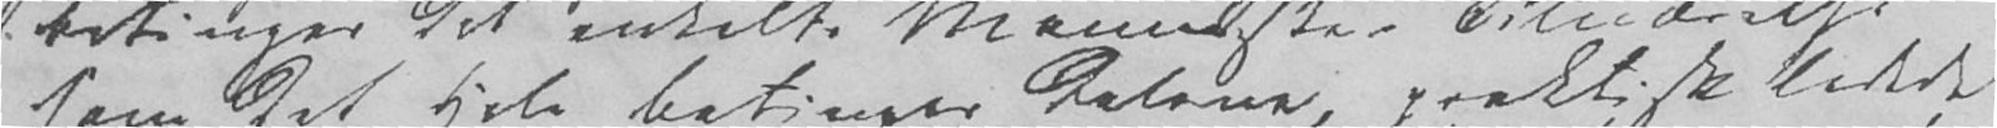som det Hele betinger Delene, praktisk ledede
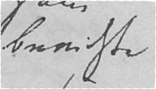bevidste
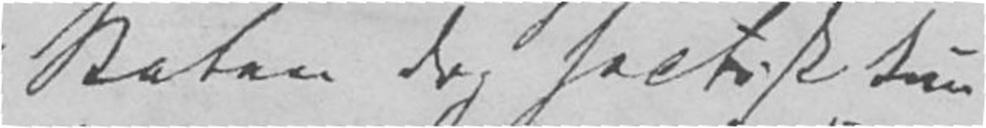Staten dog factisk kun
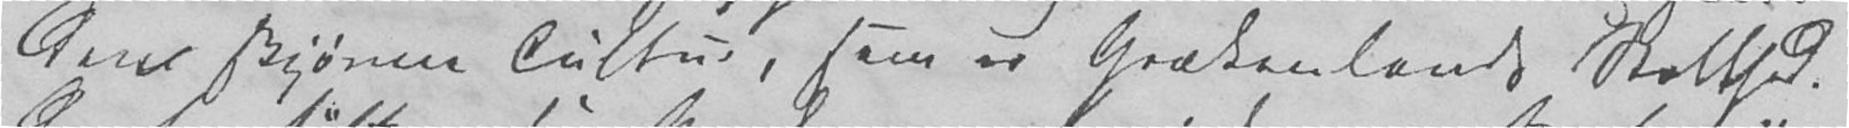den skjønne Cultur, som er Grækenlands Stolthed.
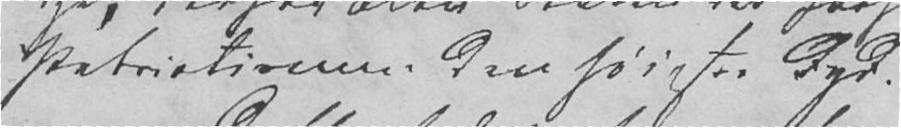Patriotismen den høieste Dyd.
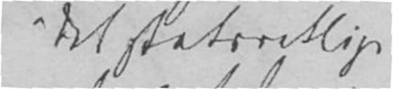det statsretlige
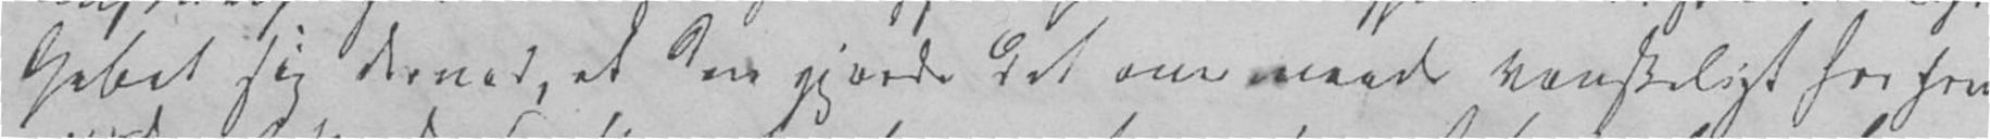Gebet sig derved, at den gjorde det over Maade vanskeligt for frem-
